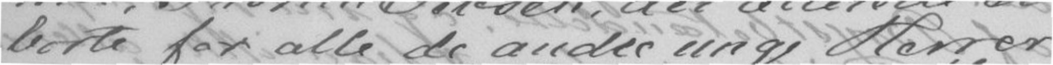borte for alle de andre unge Herrer
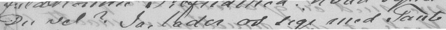Du vel? Ja, lader os sige med Tante
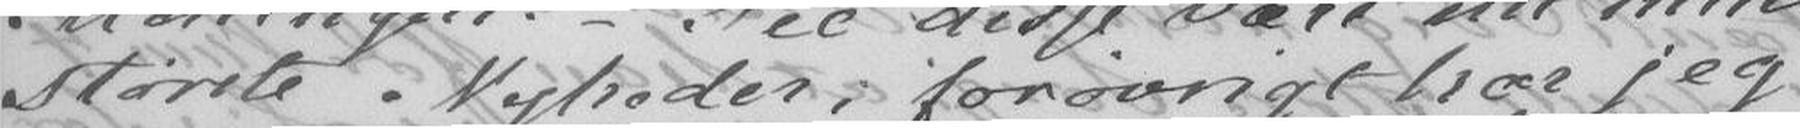største Nyheder; forøvrigt har jeg
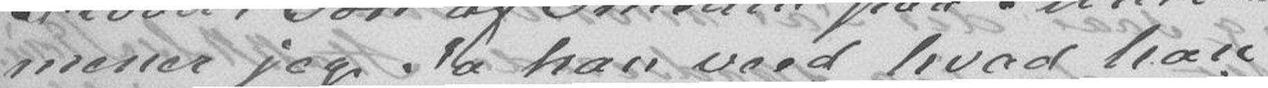mener jeg. Ja han veed hvad han
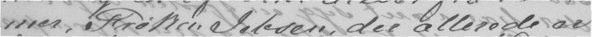mer, Frøken Jebsen, der allerede er
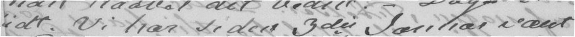lidt. Vi har siden 3die Januar været
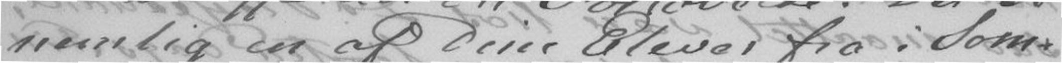nemlig en af Dine Elever fra i Som-
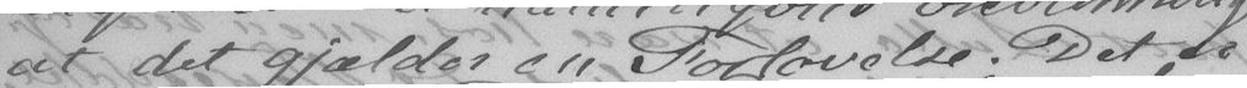at det gjælder Forlovelse. Det er
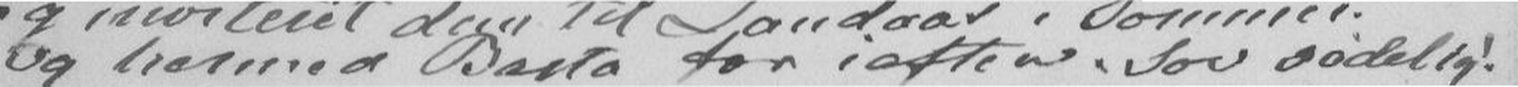Og hermed Basta for iaften. Lev sødelig!
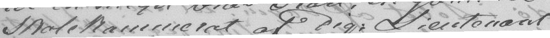Skolekammerat af Dig; Lieutenant
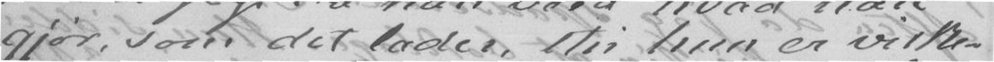gjør, som det lader, thi hun er virke-
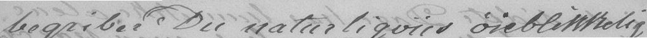begriber Du naturligviis øieblikkelig
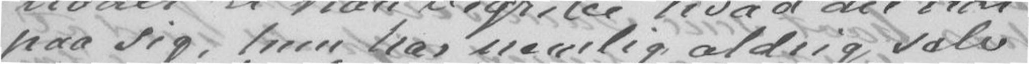paa sig, hun har nemlig aldrig selv
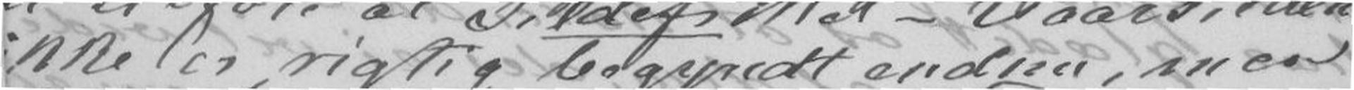ikke er rigtig begyndt endnu, men
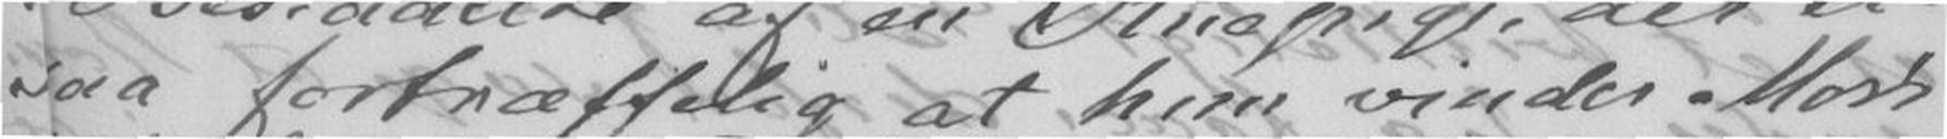saa fortræffelig at hun vinder Mors
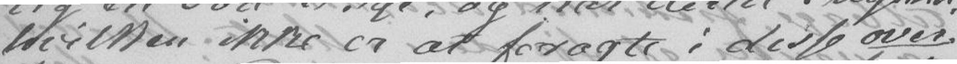Hvilken ikke er at foragte i disse over-
